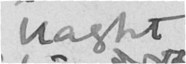Uagtet
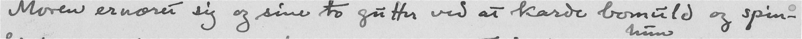Moren ernæret sig og sine to gutter ved at karde bomuld og spin-
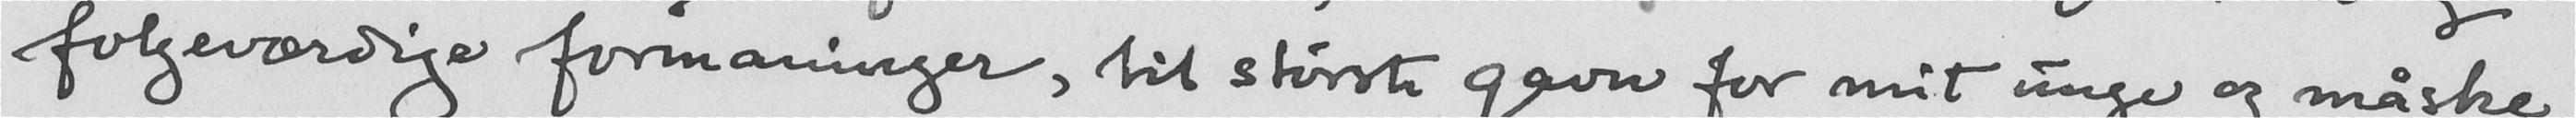folgeværdige formaninger, til største gavn for mit unge og måske
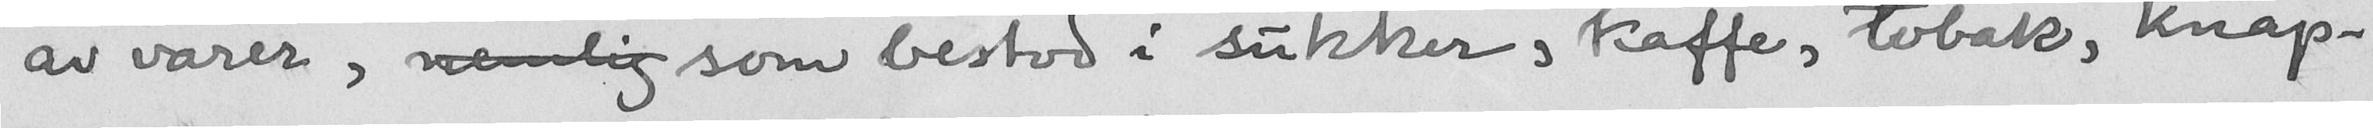av varer, nemlig "som bestod i sukker, kaffe, tobak, knap-
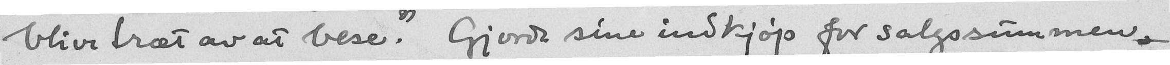blive træt av at bese." Gjorde sine indkjøp for salgssummen,
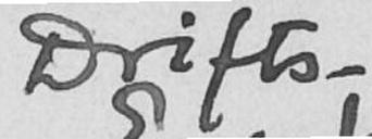Drifts-
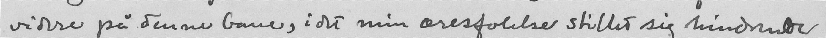videre på denne bane, idet min æresfølelse stillet sig hindrende
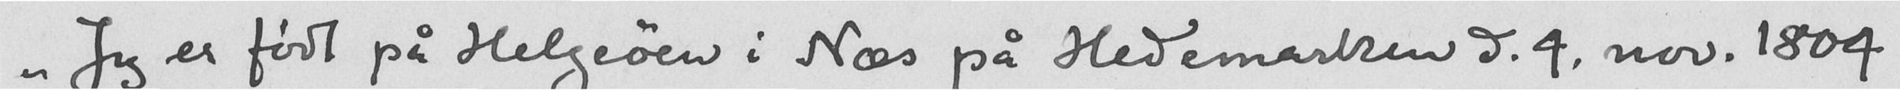"Jeg er født på Helgeøen i Næs på Hedemarken d. 4. nov. 1804
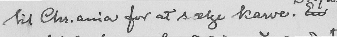til Chr.ania for at sælge karve. En
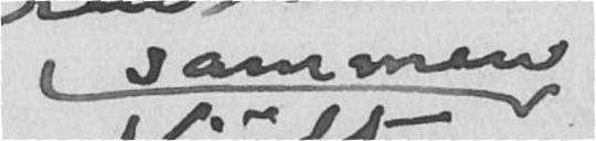sammen
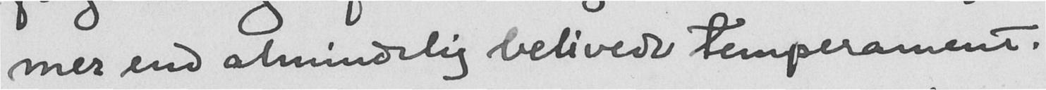mer end almindelig belivede temperament."
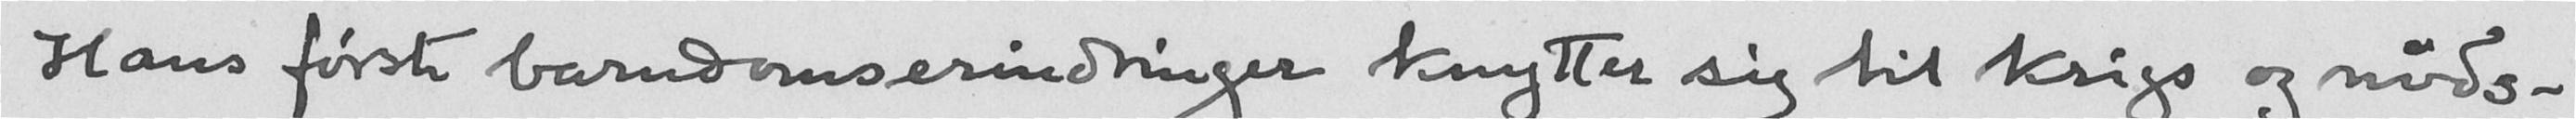Hans første barndomserindringer knytter sig til krigs og nøds-
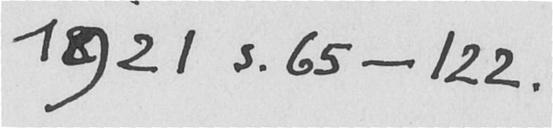1921 s.65 - 122.
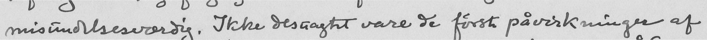misundelsesværdig. Ikke desuagtet vare de første påvirkninger af
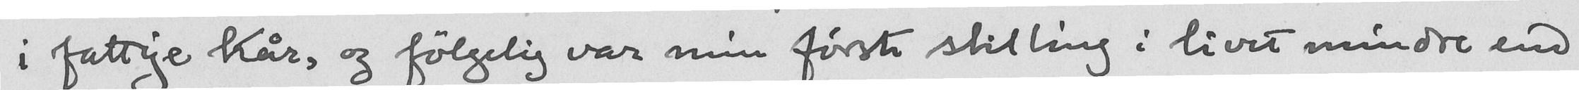i fattige kår, og følgelig var min første stilling i livet mindre end
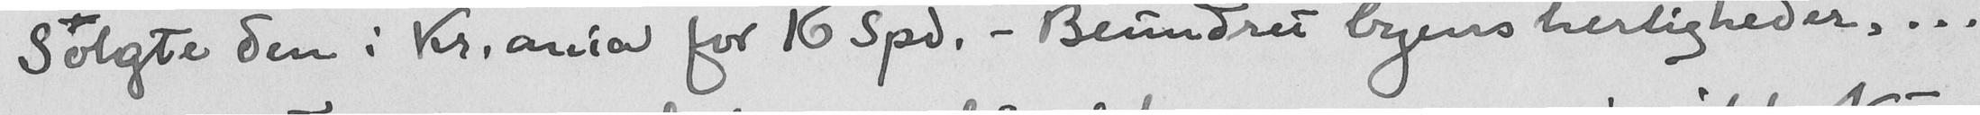Solgte den i Kr.ania for 16 Spd. - "Beundret byens herligheder,...
